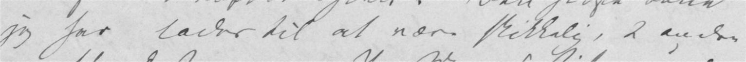jeg har lader til at være skikkelig, 2 andre
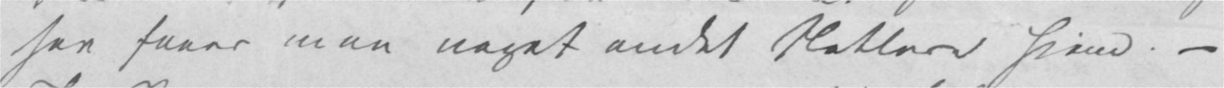saa faaer man noget andet Slettere hjem. –
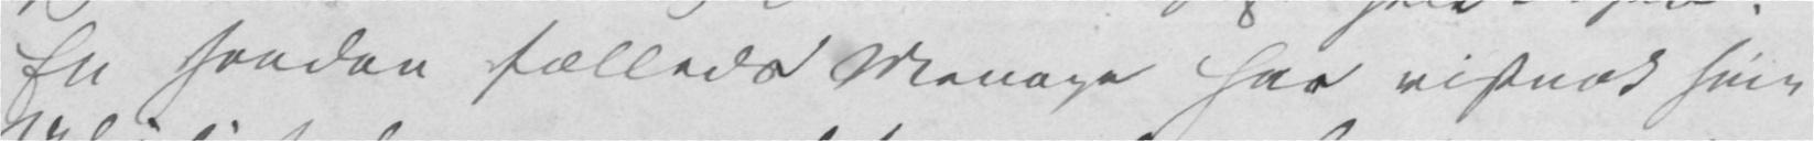En saadan fælleds Menage har vistnok sine
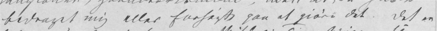bedraget mig eller forsøgte paa at gjøre det. Det er
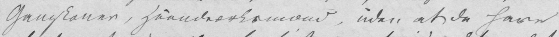Gangkoner, Haandværksmænd, uden at de have
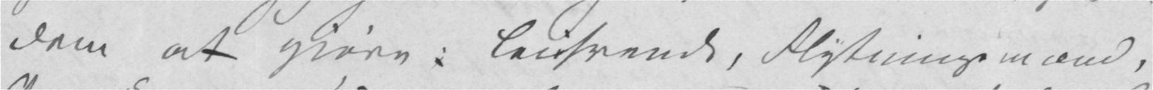dem at gjøre: Leiesvende, Flytningsmænd,
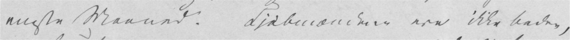eneste Maaned. Kjøbmændene ere ikke bedre,
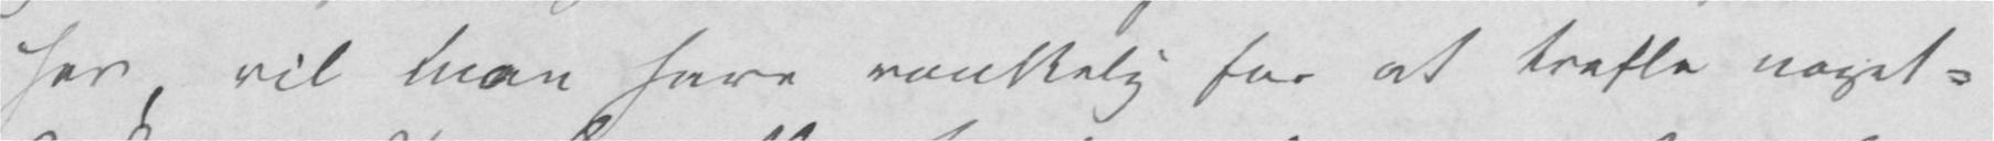her, vil man have vanskelig for at treffe noget-
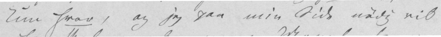kun hvor, og jeg paa min Side nødig vil
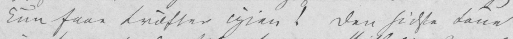kun faae Kræfter igjen! Den sidste Kone
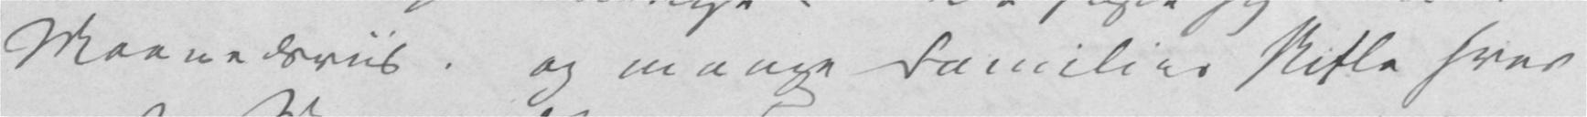Maanedsviis. og mange Familier skifte hver
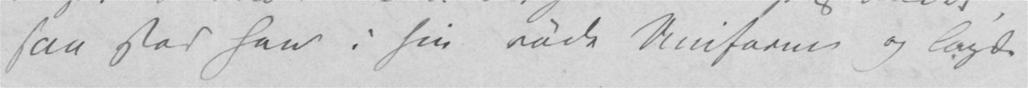saa stod han i sin røde Uniform og lagde
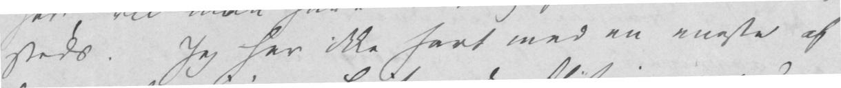steds. Jeg har ikke havt med en eneste af
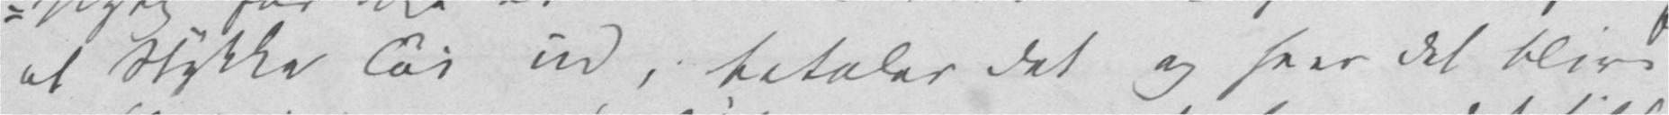et Stykke Tøi ud, betaler det og seer det blive
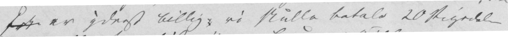er yderst billig: vi skulle betale 20 Rigsdaler
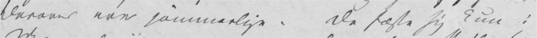derover ere jammerlige. De fæste sig kun i
